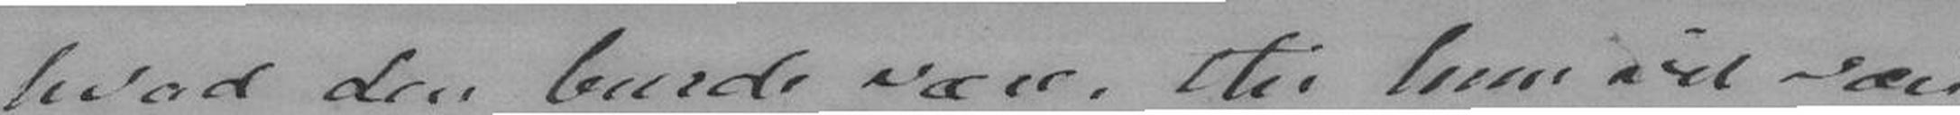hvad burde Være, thi hun vil være
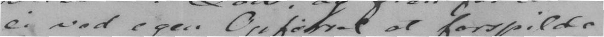ei ved egen Opførsel at forespilde
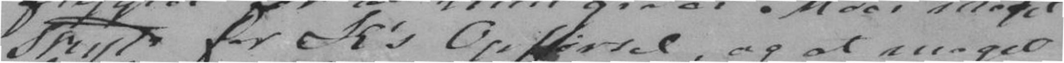Skyld for K's Opførsel, og at meget
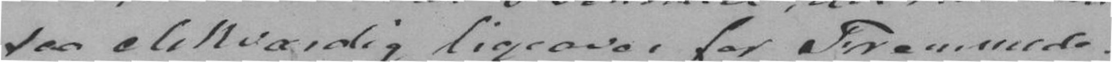saa elskværdig ligeover for Fremmede,
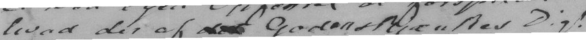hvad der af det Goder skjænkes Dig!
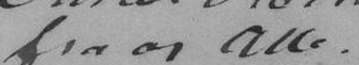fra os Alle.
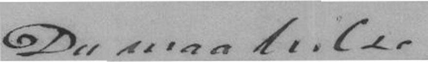Du maa hilse
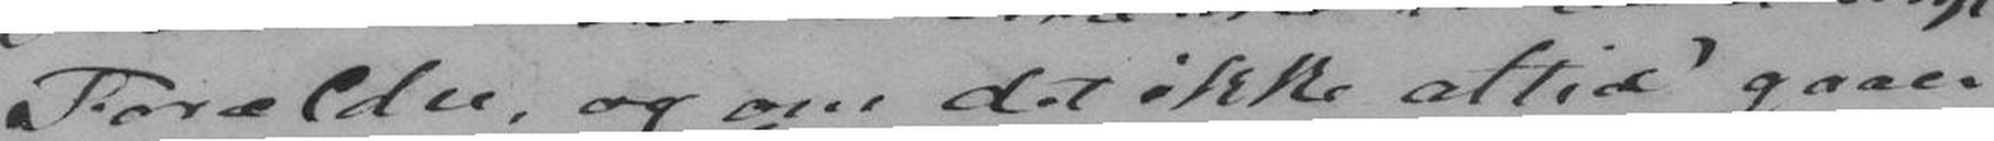Forældre, og om det ikke altid gaaer
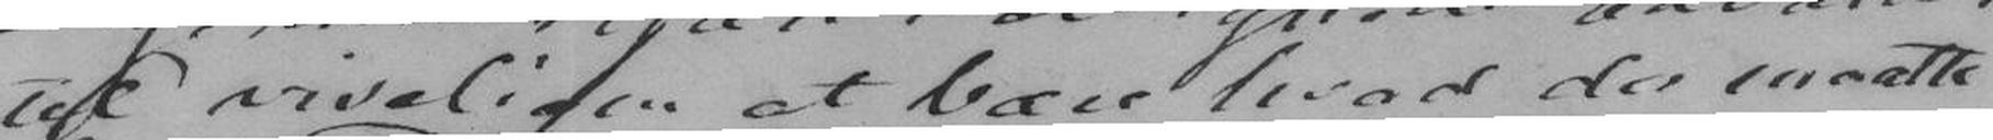til viseligen at bære hvad der maatte
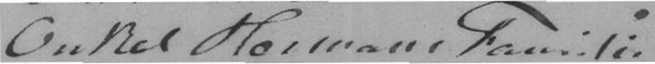Onkel Hermans Familie
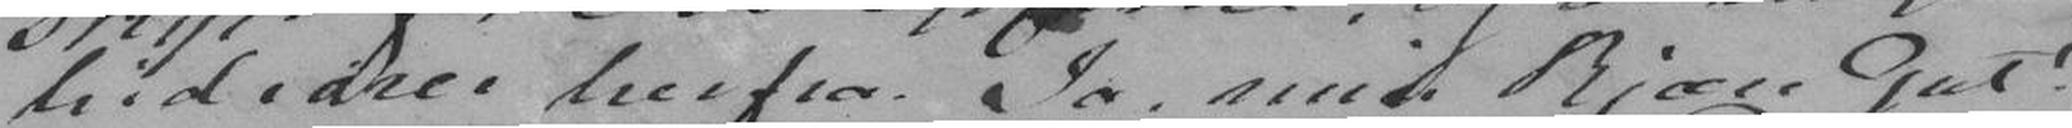hid varer herfra. Ja, min kjære Gut!
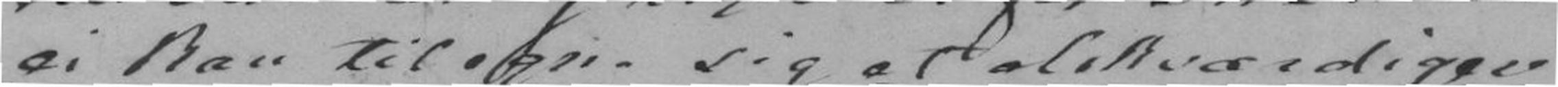ei kan tilegne sig et elskværdigere
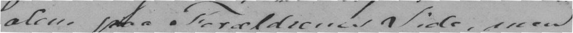alene paa Forældrenes Side, men
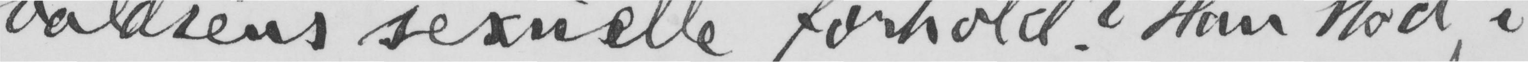valdsens sexuelle forhold? Han stod i
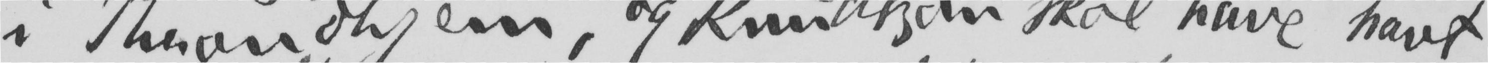i Throndhjem, og Knudtzon skal have havt
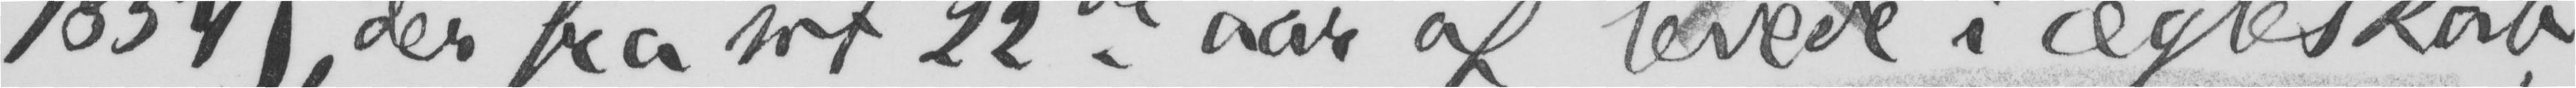1854), der fra sit 22de aar af levede i ægteskab
